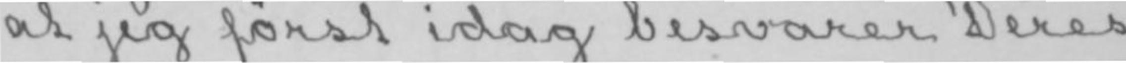at jeg først idag besvarer Deres
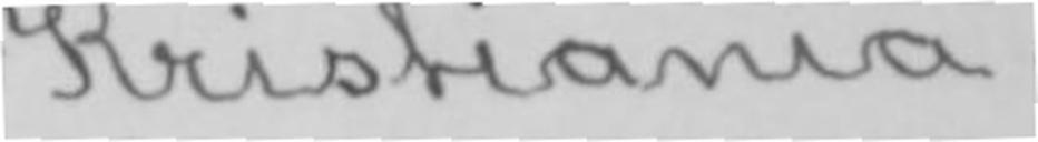Kristiania.
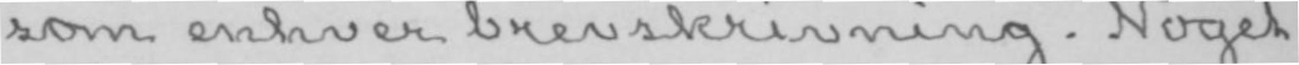som enhver brevskrivning. Noget
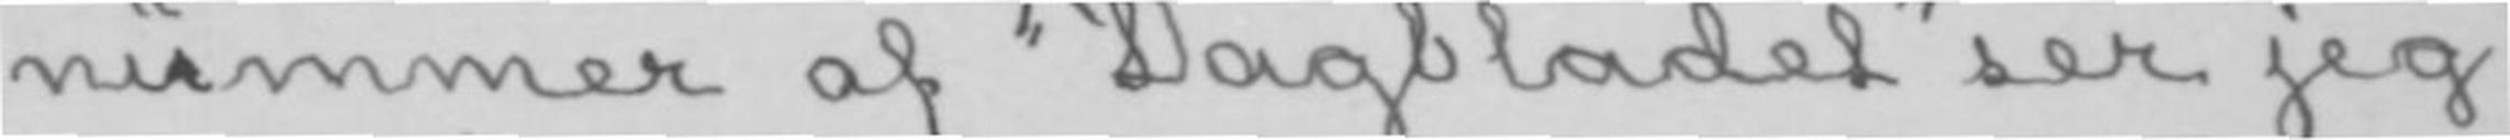nummer af «Dagbladet» ser jeg
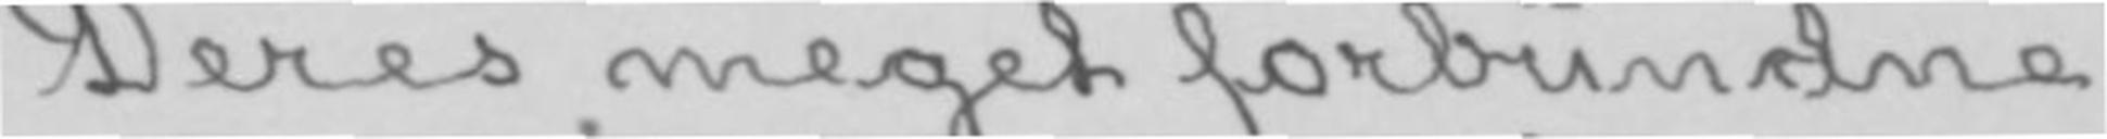Deres meget forbundne
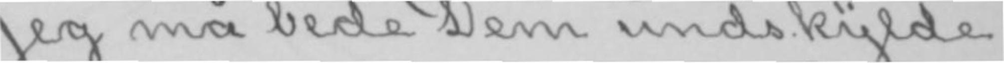Jeg må bede Dem undskylde
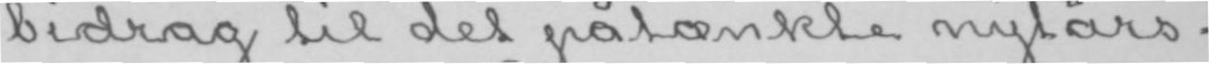bidrag til det påtænkte nytårs-
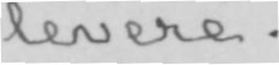levere.
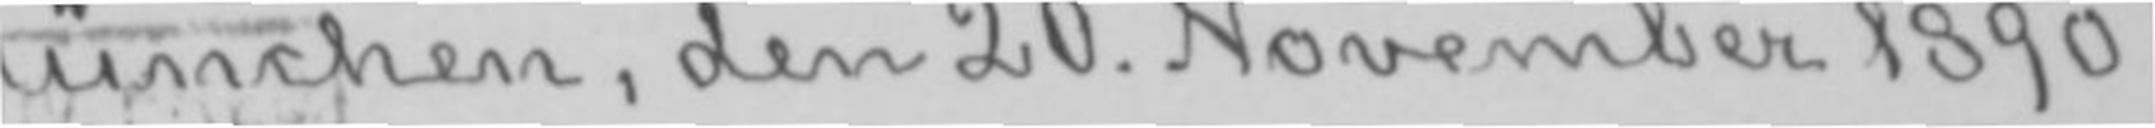München, den 20. November 1890.
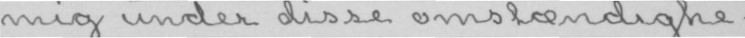mig under disse omstændighe-
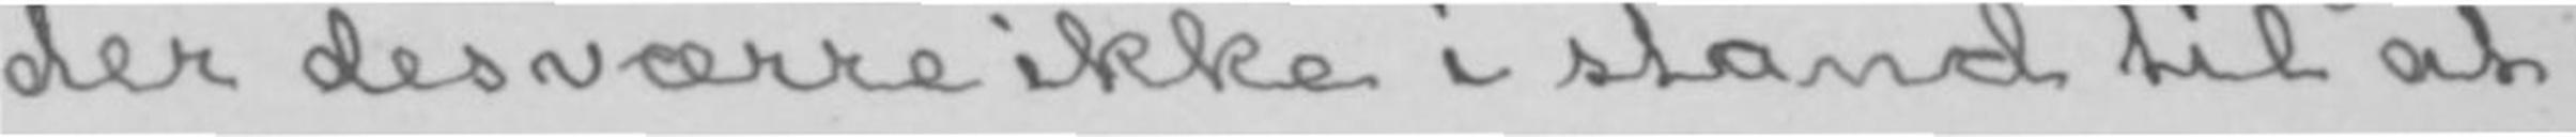der desværre ikke i stand til at
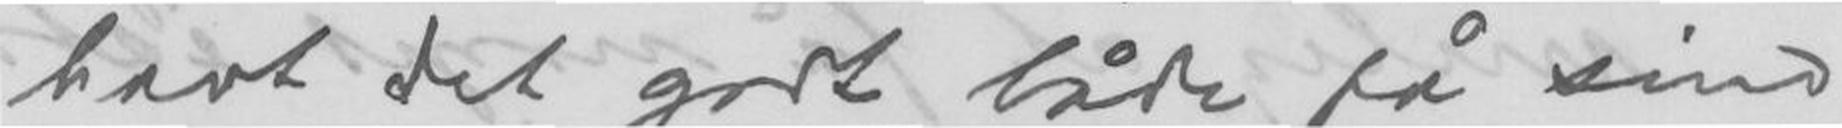havt det godt både på sind
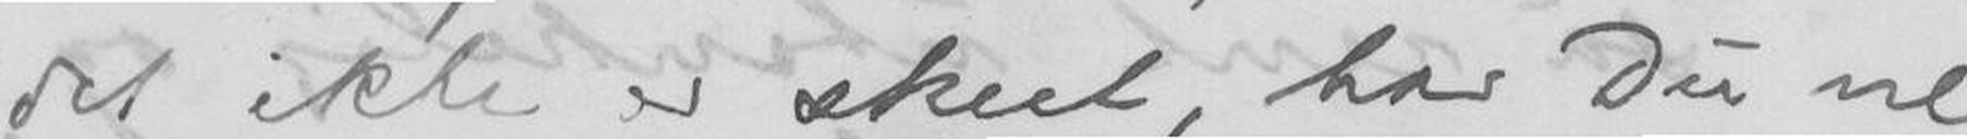det ikke er skeet, har Du vel
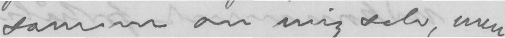samme om mig selv, men
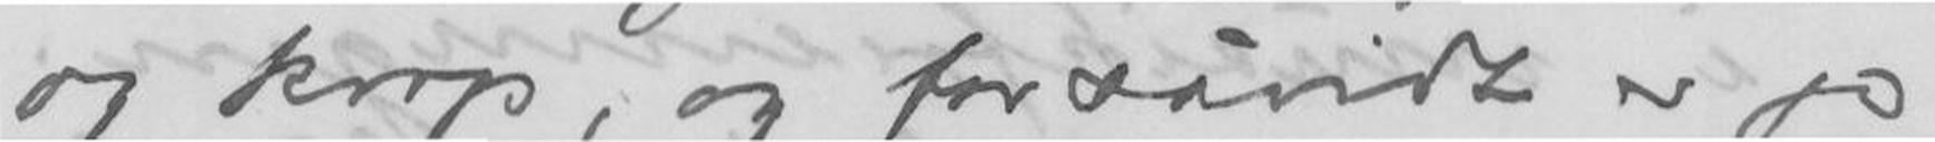og krop, og for såvidt er jo
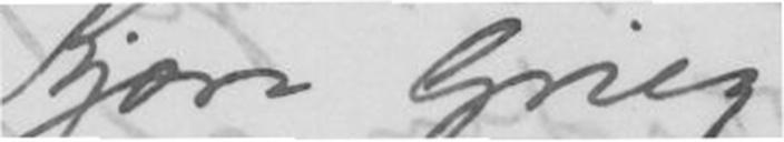Kjære Grieg!
